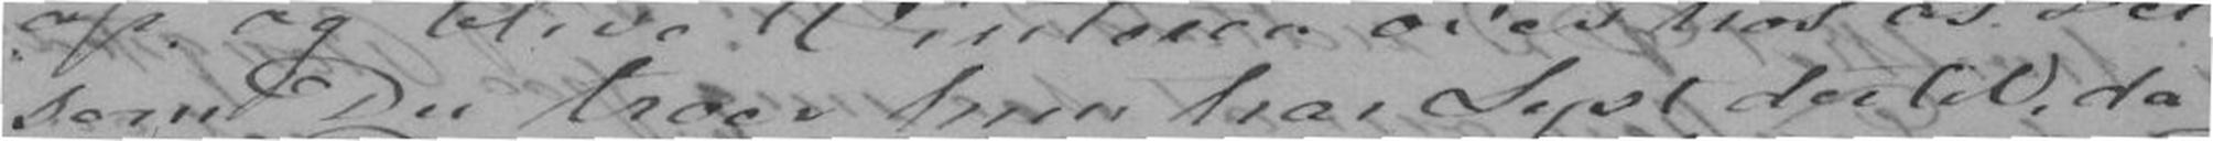som Du troer hun har Lyst dertil, da
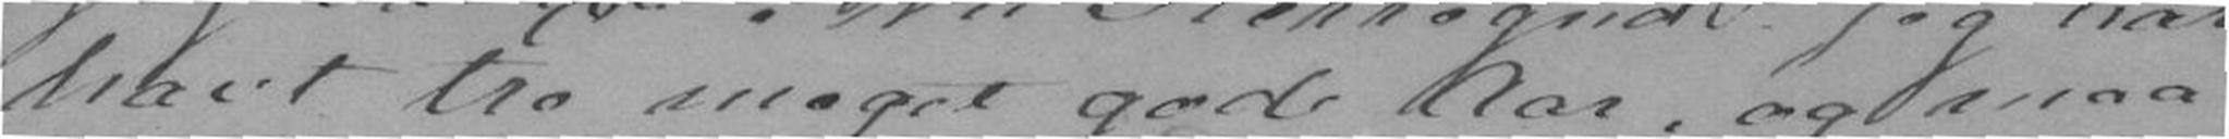havt tre meget gode Aar, og maa
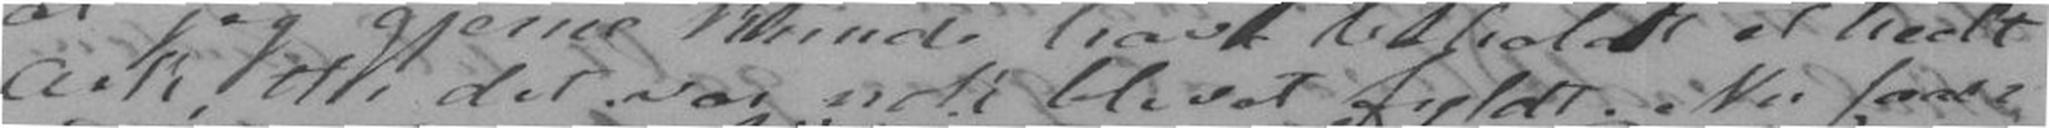Ark, thi det var nok blevet fyldt. Nu faae
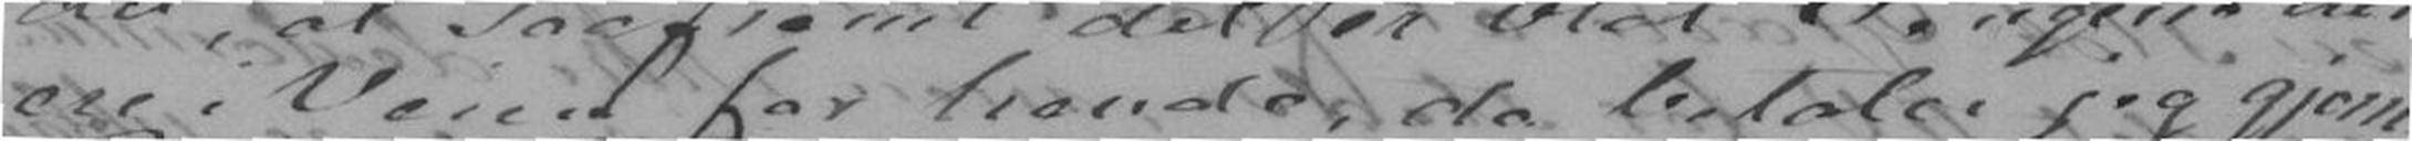ere i Veien for hende, da betaler jeg gjerne
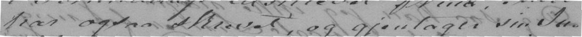har ogsaa skrevet, og gjentager sin In-
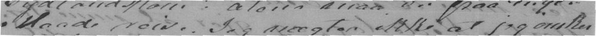Maade reise. Jeg nægter ikke at jeg ønsker
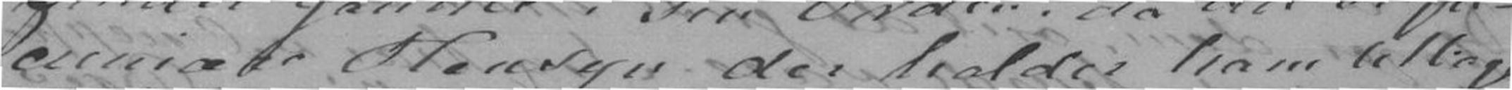cuniære Hensyn der holder ham tilbage.
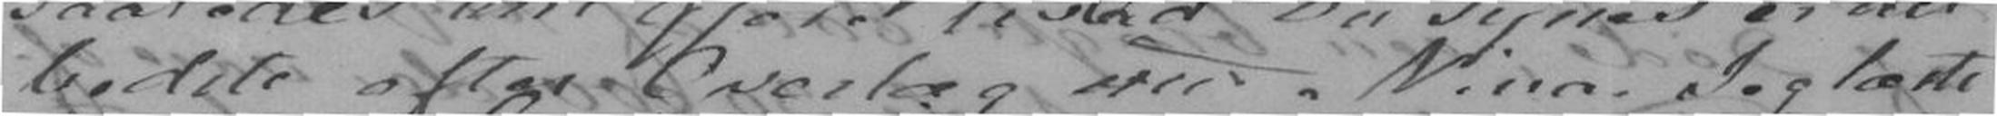bedste efter Overlæg med Nina. Jeg læste
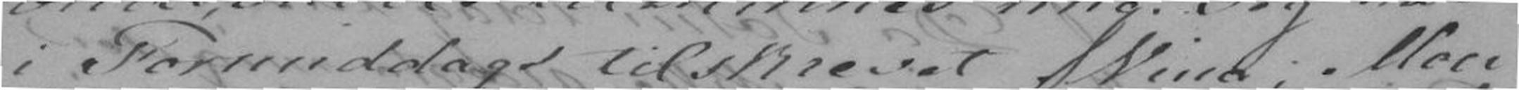i Formiddag tilskrevet Nina, Moer
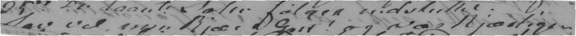Lev vel min kjære Gut! og vær kjærligen
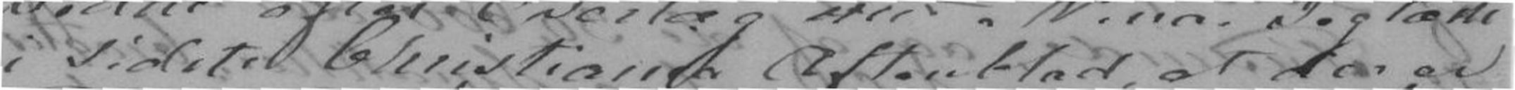i sidste Christiania Aftenblad, at der er
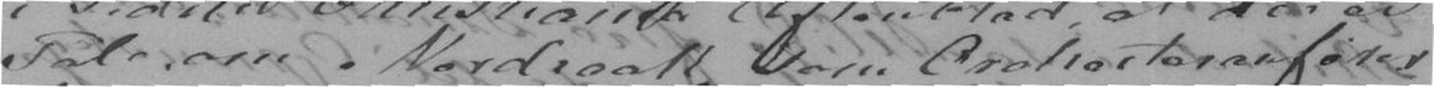Tale om Nordraak som Orchesteranfører
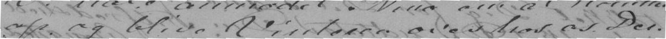op og blive Vinteren over hos os. Der-
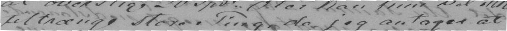tiltrenge store Ting, da jeg antager at
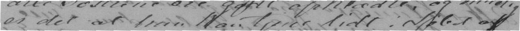er det at hun kan tjene lidt i Løbet af
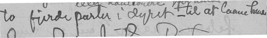to fjordeparter i dyret - til at laane hende
